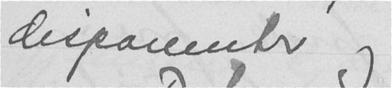disponenter og
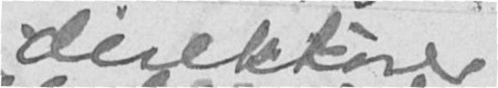direktører
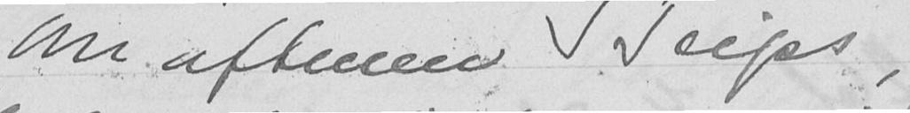Om aftenen Seips,
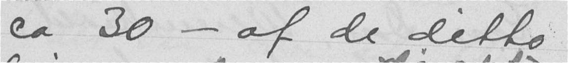ca 30 - af de ditto
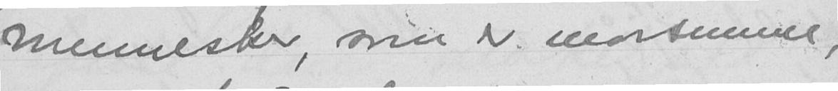mennesker, som er morsomme,
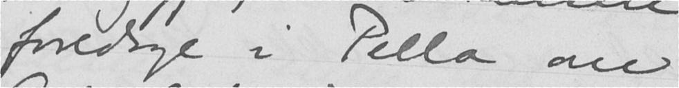foredrage i Pella om
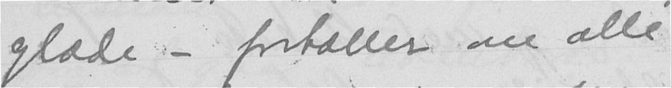glæde - fortæller om alle
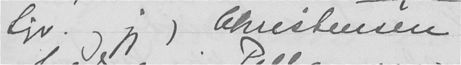Sigr. og jeg) Christensen
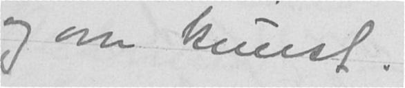og om kunst.
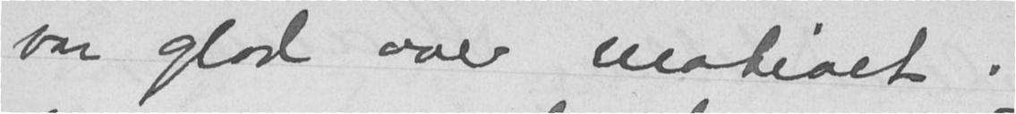var glad over motivet.
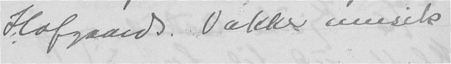Hofgaards. Vakker musik
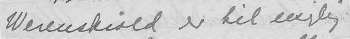Werenskiold er til usigelig
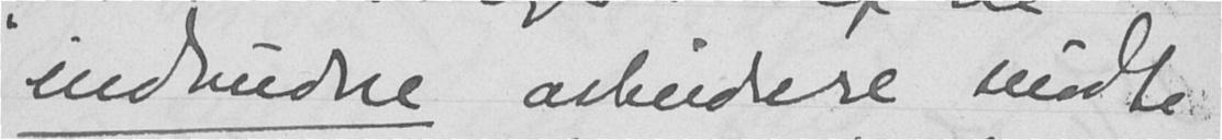indbudne arbeidere mødte
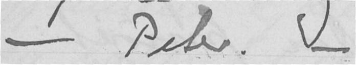- Peter. -
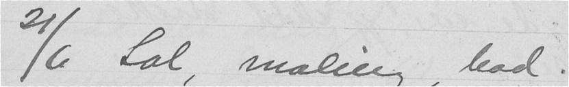21/6 Sol, maling, bad.
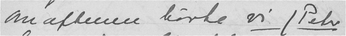Om aftenen hørte vi (Peter
